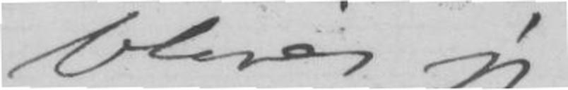bliver jeg
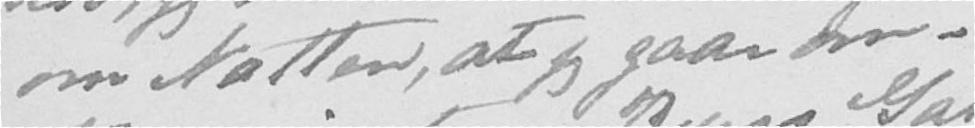om Natten, at jeg gaar om-
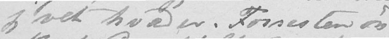jeg vet hvad er. Forresten øn-
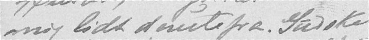mig lidt derutefra. Gud ske
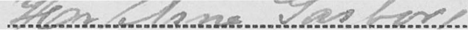Hr. Arne Garborg
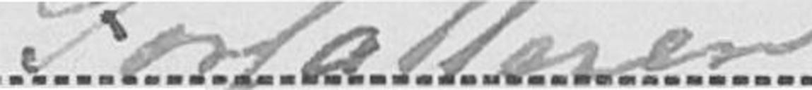Forfatteren
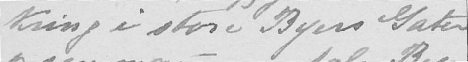kring i store Byers Gater
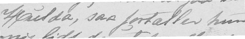Hulda, saa fortæller hun
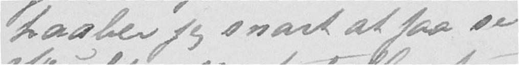haaber jeg snart at faa se
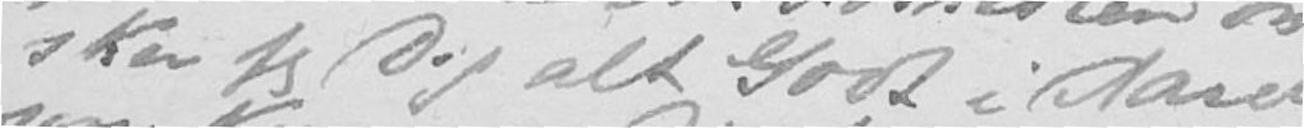sker jeg Dig alt Godt i Aaret
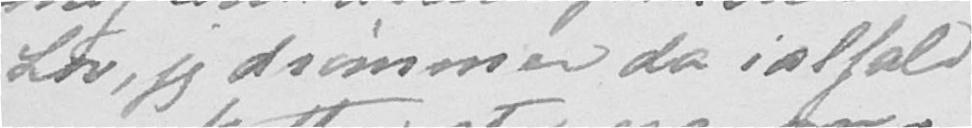Lov, jeg drømmer da ialfald
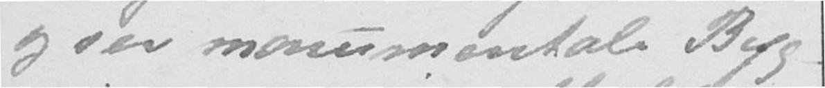og ser monumentale Byg-
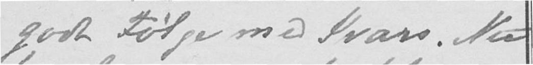godt Følge med Ivars. Nu
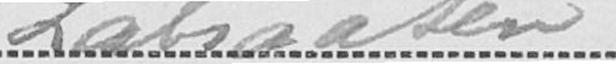Labraaten
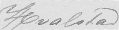Hvalstad
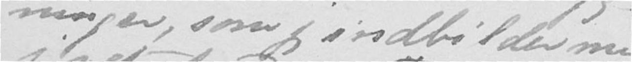ninger, som jeg indbilder mig.
